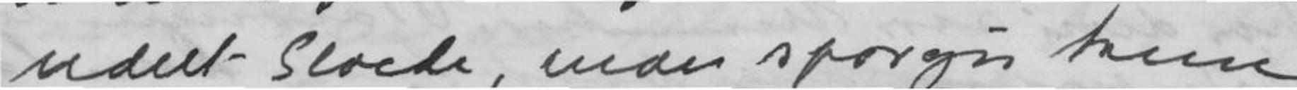udelt Slaede, Man spørger kun
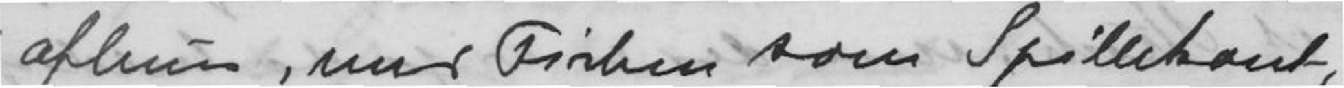-aftners, med Fisken som Spillekant,
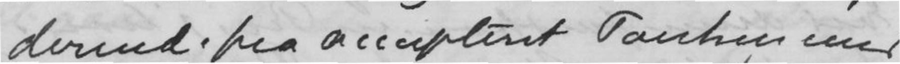derved paa accupteret Tanken med
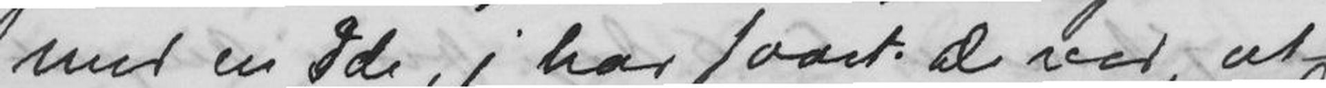med en Ide, j har faaet De ved, at
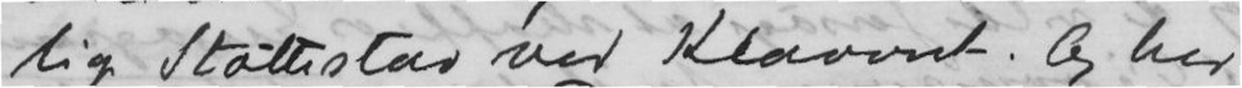lig Støttestav ved Klaveret. Og her
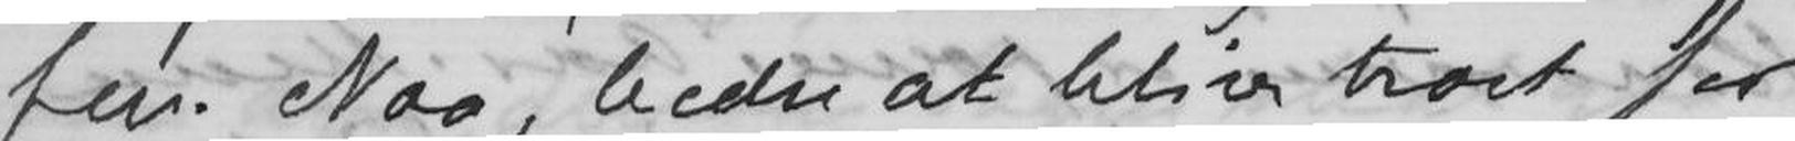fen. Naa, bedre at blive troet for
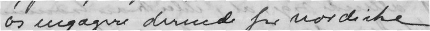os engagere dernede for nordiske
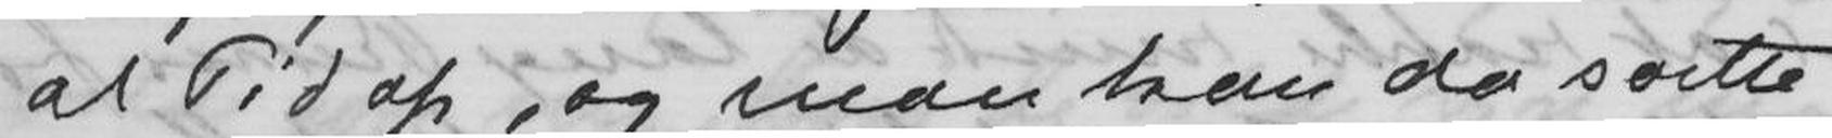al Tid op, og man kan da saette
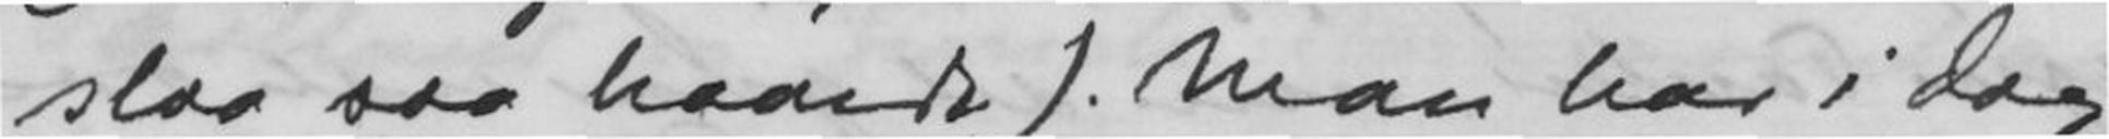slaa saa haardt). Man har i dag
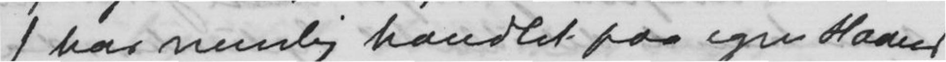J har nemlig handlet paa egen Haand
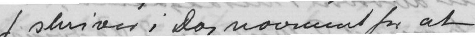J skriver i Dag naermest for at
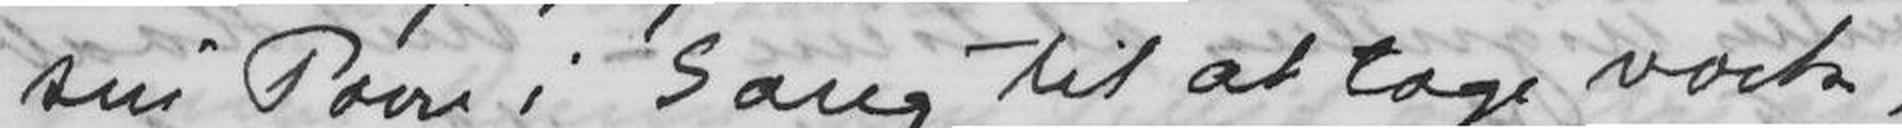sin Poesi i Sang til at tage vaek,
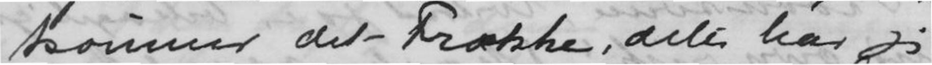kommer det Frække, dilis har jeg
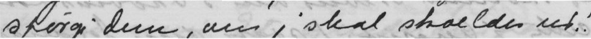spørge Dem, om j skal skaeldes ud!!
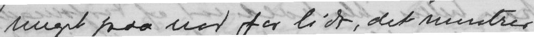meget paa end for lidt, det mundrer
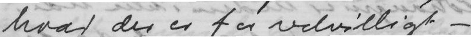hvad der er for velvilligt -.-
